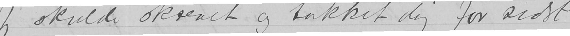Jeg skulde skrevet og takket dig for sidst
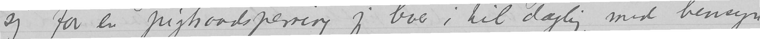sig for en pigtraadsperring jeg lever boer i til daglig, med hensyn
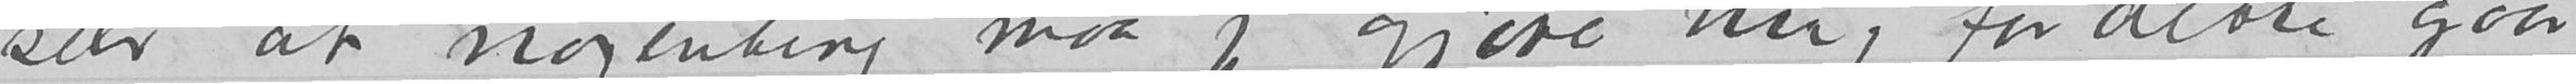selv at nogenting maa jeg gjøre nu, for dette
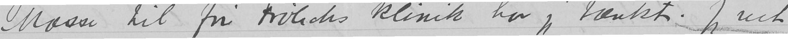Mosse til fru Frølichs klinik har jeg tænkt. Jeg vet
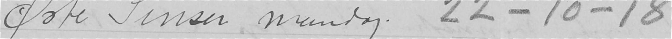Østre Sinsen mandag.
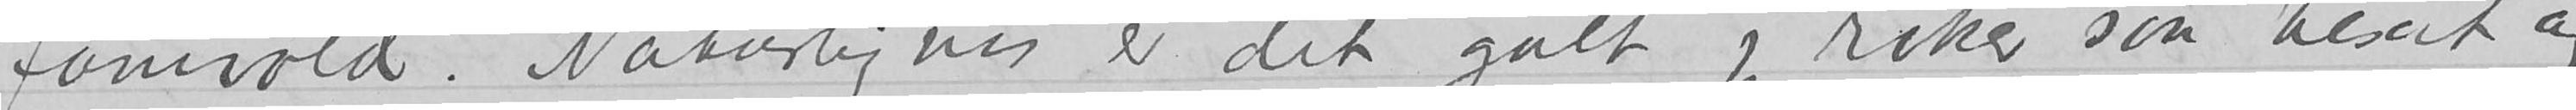gaar fanivold. Naturligvis er det galt jeg røker som besat og,
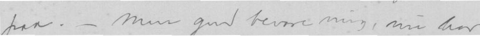paa.- Men gud bevare mig, nu har
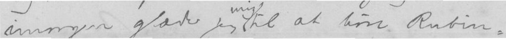imorgen glæder jeg til at høre Rubin-
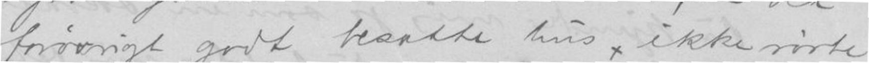forøvrigt godt besatte hus, ikke rørte
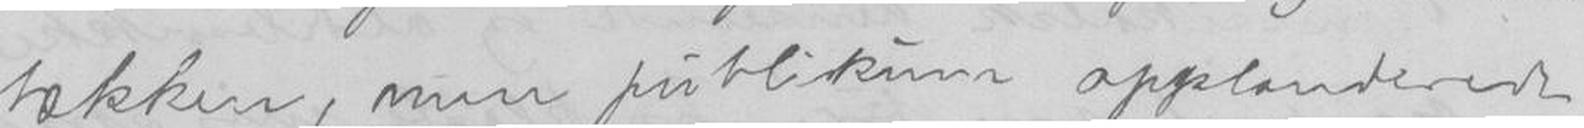tækken, men publikum applauderede
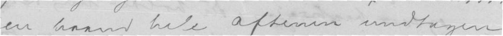en haand hele aftenen undtagen
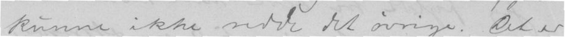kunne ikke redde det øvrige. Det er
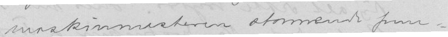maskinmesteren stormende frem-
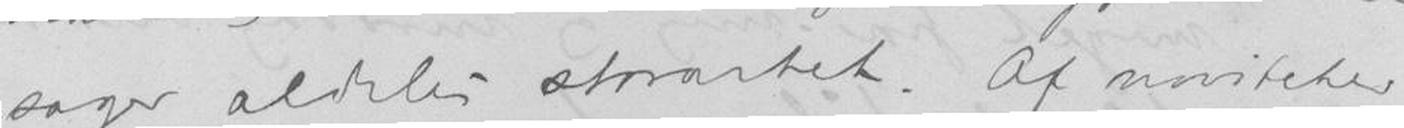sager aldeles storartet. Af noviteter
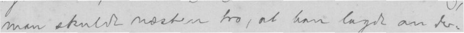man skulde næsten tro, at han lagde an der
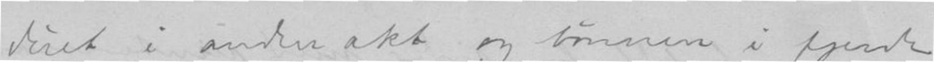duet i andre akt og bønnen i fjerde
